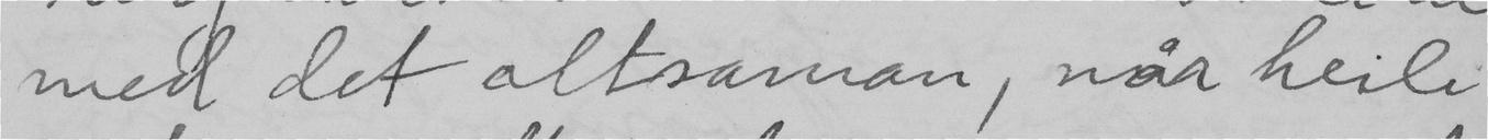med det altsaman, når heile
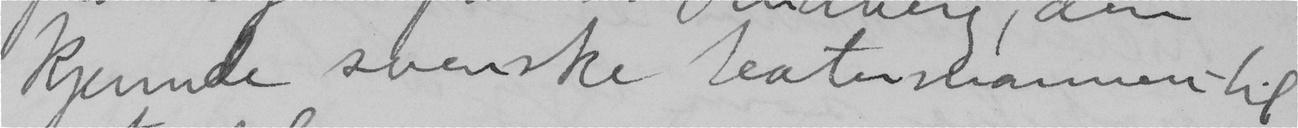kjennde svenske teatersmannen til
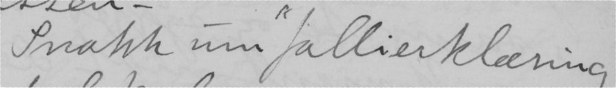Snakk om "falliterklæring"–
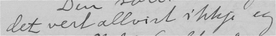det vert allvist ikkje eg –
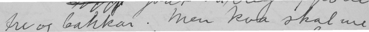tre og bakkar. Men kva skal me
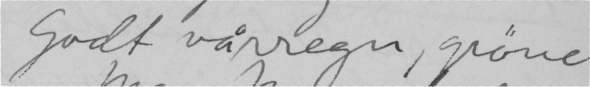Godt vårregn, gröne
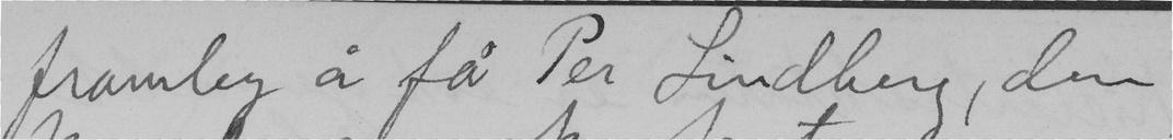framleg å få Per Sindberg, den
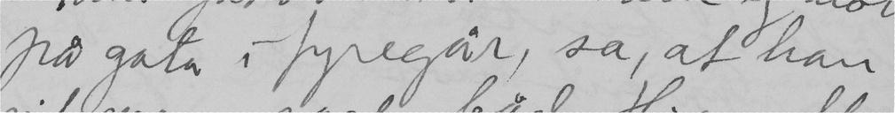på gata i fyregår, sa, at han
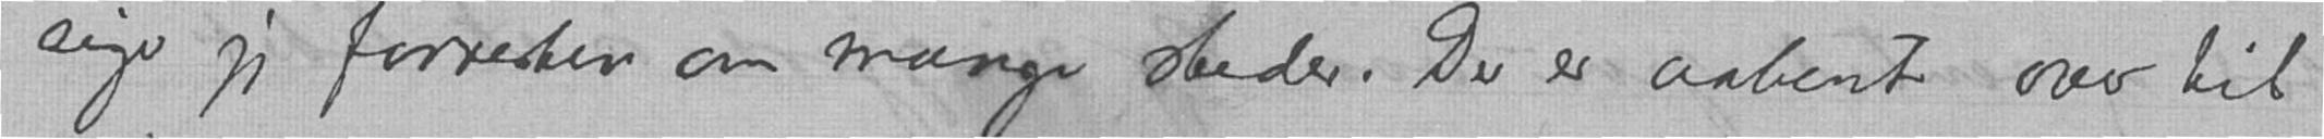siger jeg forresten om mange steder. Der er aabent over til
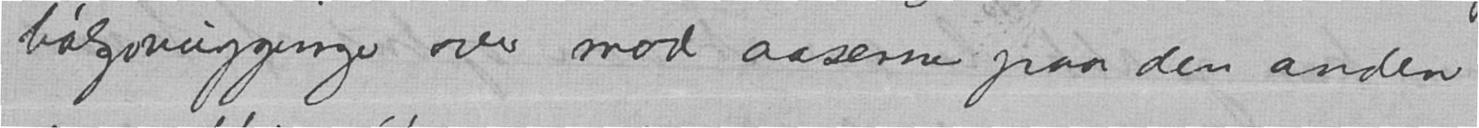bølgevugginger over mod aaserne paa den anden
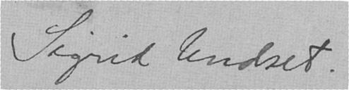Sigrid Undset.
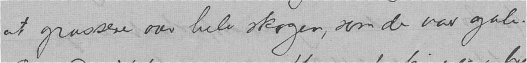at grassere over hele skogen, som de var gale. -
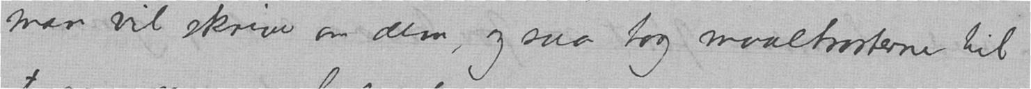man vil skrive om dem, og saa tog maaltrosterne til
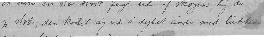jeg stod, den kastet sig ud i dybet under med lukkede
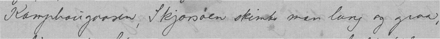Kamphaugaasen, Skjærsøen skimter man lang og graa,
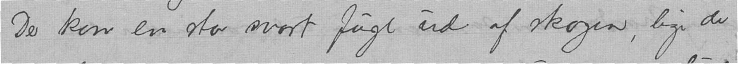Der kom en stor svart fugl ud af skogen, lige der
